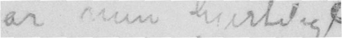far min hjertelige
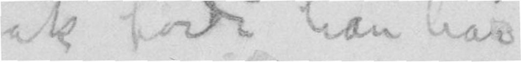tak fordi han har
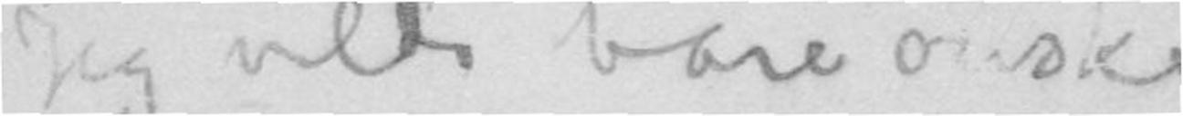Jeg vilde bare ønske
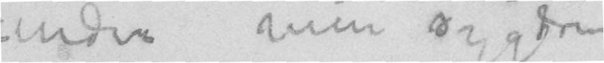under min sygdom
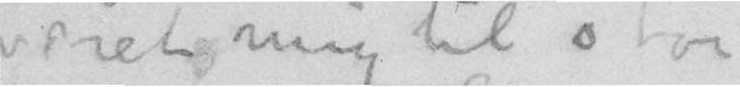været mig til stor
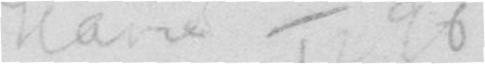Havre /12 96
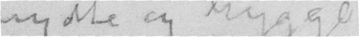nydte og hygge
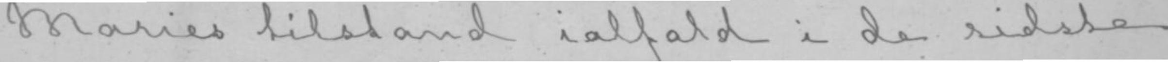Maries tilstand ialfald i de sidste
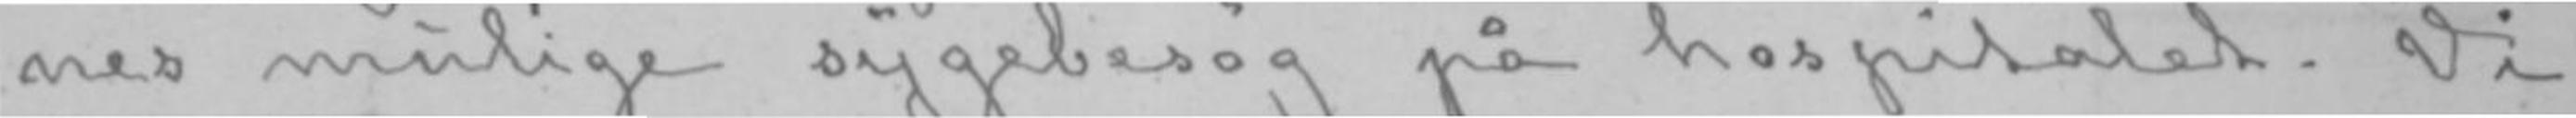nes mulige sygebesøg på hospitalet. Vi
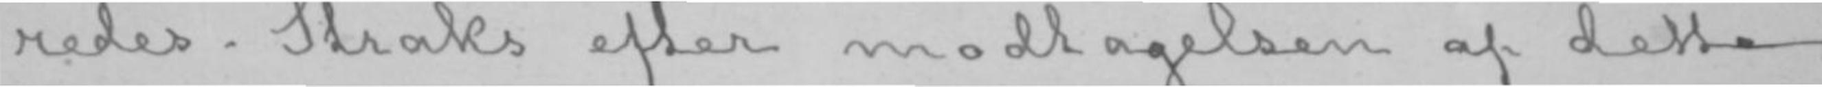redes. Straks efter modtagelsen af dette
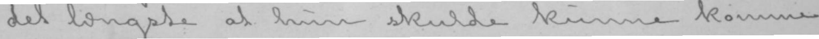det længste at hun skulde kunne komme
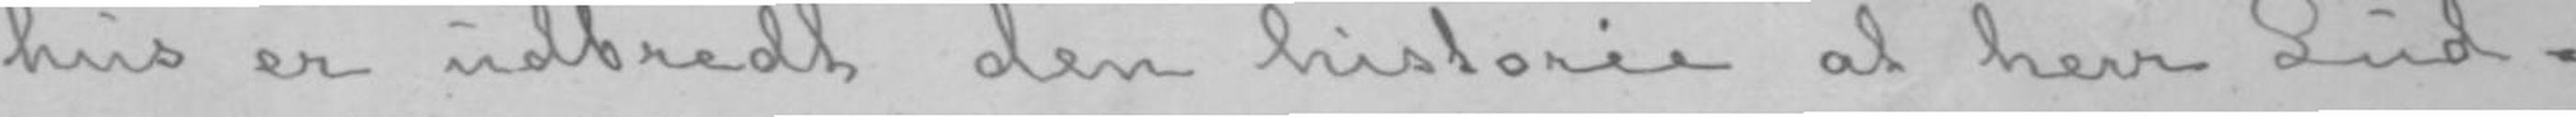hus er udbredt den historie at herr Lud-
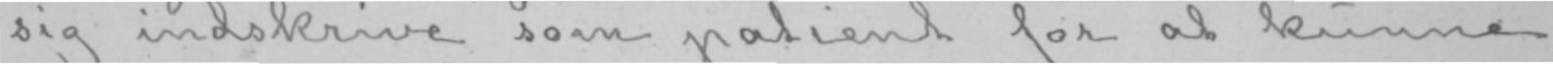sig indskrive som patient for at kunne
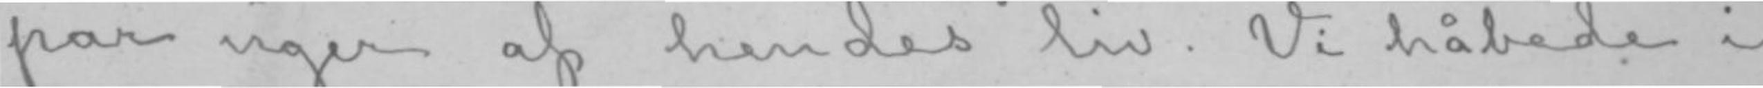par uger af hendes liv. Vi håbede i
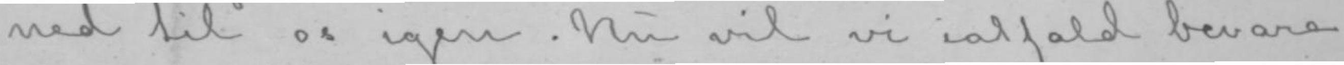ned til os igen. Nu vil vi ialfald bevare
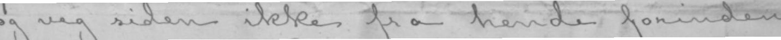og veg siden ikke fra hende forinden
